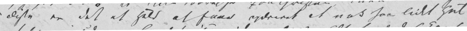disse er det et Held at faae opdrevet et nok saa lidet Hul
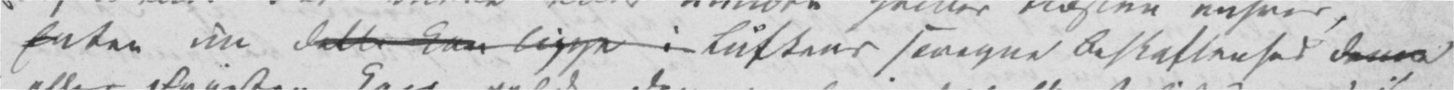enten nu dette kan ligge i Luftens særegne Beskaffenhed denne
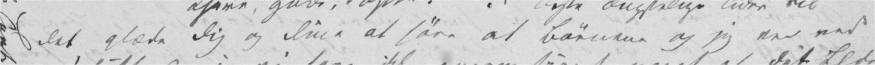det glæde Dig og Dine at høre at Børnene og jeg ere ved
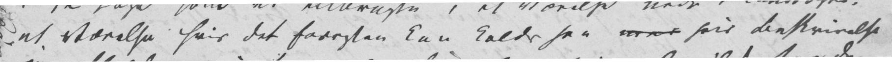et Værelse hvis det forresten kan kaldes saa mer hvis Beskrivelse
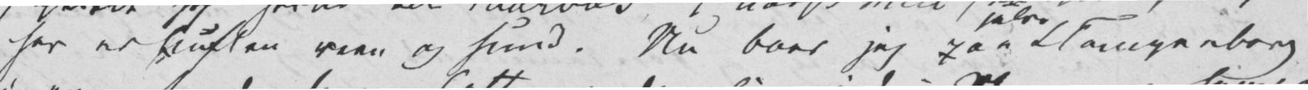her er Luften reen og sund. Nu boer jeg paa Klampenborg
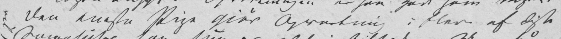Den eneste Pige gjør Opvartning i flere af disse
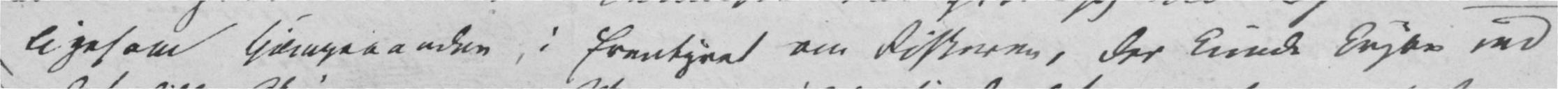ligesom Kjæmpeaanden i Eventyret om Fiskeren, der kunde krybe ind
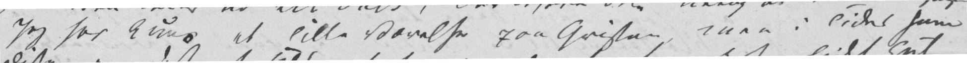Jeg har kuns et lille Værelse paa Qvisten, men i Tider som
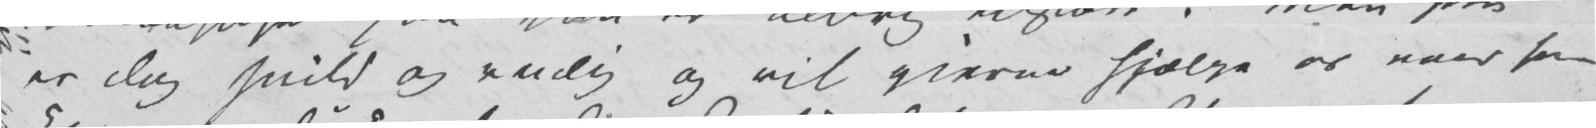er dog snild og venlig og vil gjerne hjælpe os naar hun
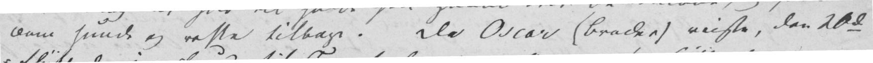dem sunde og raske tilbage. Da Oscar (Broder) reiste, den 20de
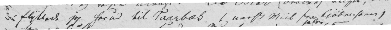flyttede jeg herud til Taarbæk, (norsk Miil fra Kjøbenhavn)
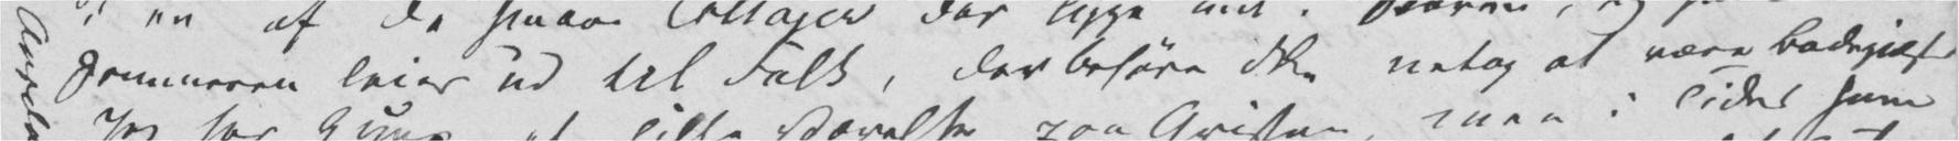Sommeren leies ud til Folk, der behøve ikke netop at være Badegjæster
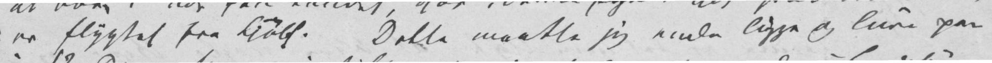er flygtet fra Kjøbh. Dette maatte jeg enda ligge og lure paa
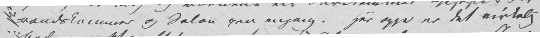raadskammer og Salon paa engang. Her oppe er det virkelig
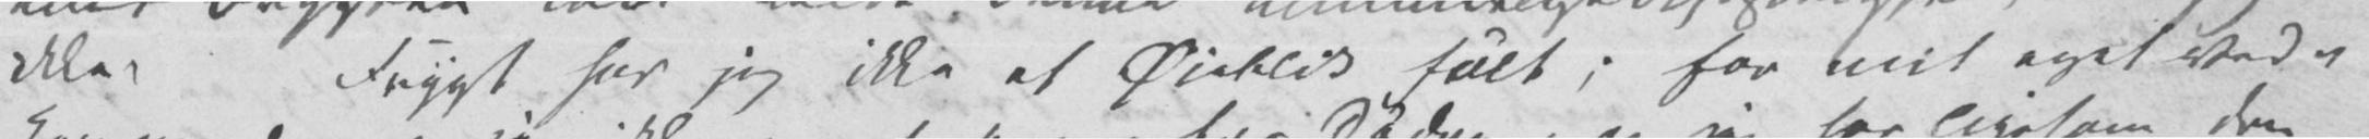ikke. Frygt har jeg ikke et Øieblik følt; for mit eget Ved-
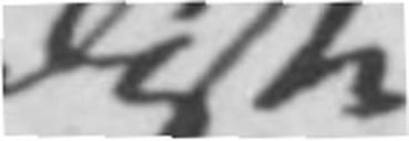disse
